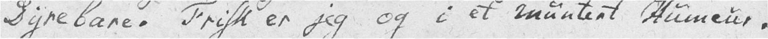Dyrebare. Frisk er jeg og i et muntert Humeur.
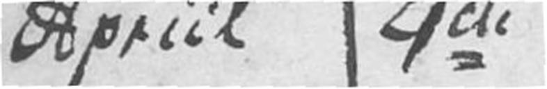Apriil 4de
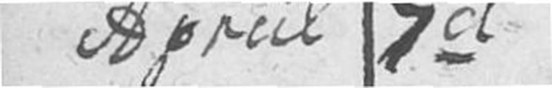Apriil 7d
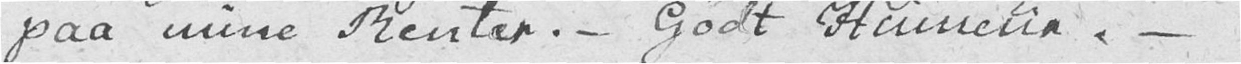paa mine Renter. – Godt Humeur. –
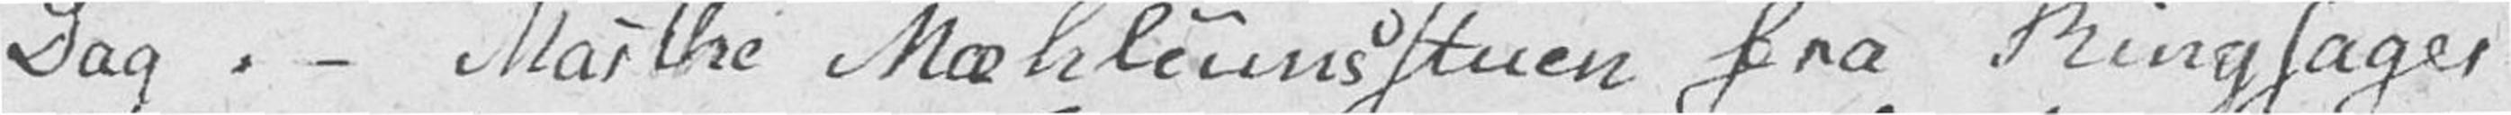Dag. – Marthe Mæhlumsstuen fra Ringsager
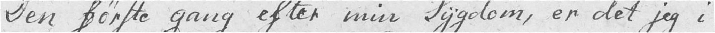Den første gang efter min Sygdom, er det jeg i
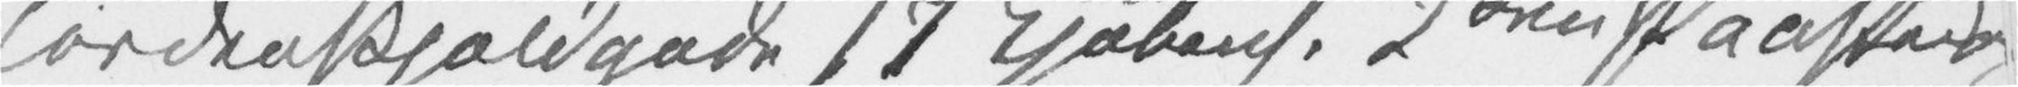Tordenskjoldgade 17 Kjøbenh. 2den Paaskedag
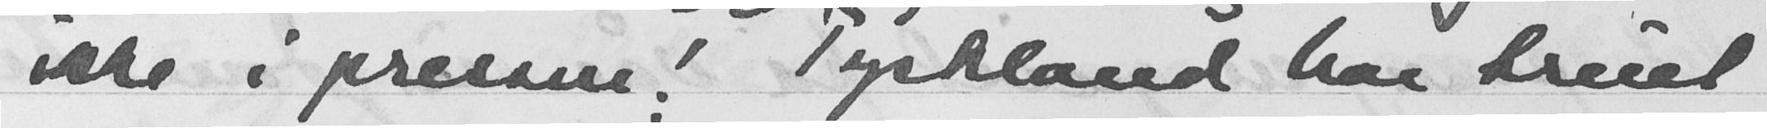ikke i pressen! Tyskland har truet
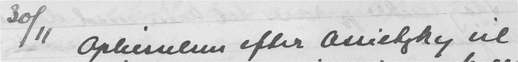30/11 Ophisselsen efter Ossietzky vil
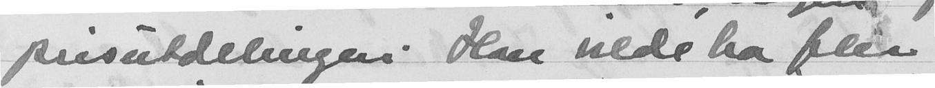prisutdelingen. Han vilde ha flere
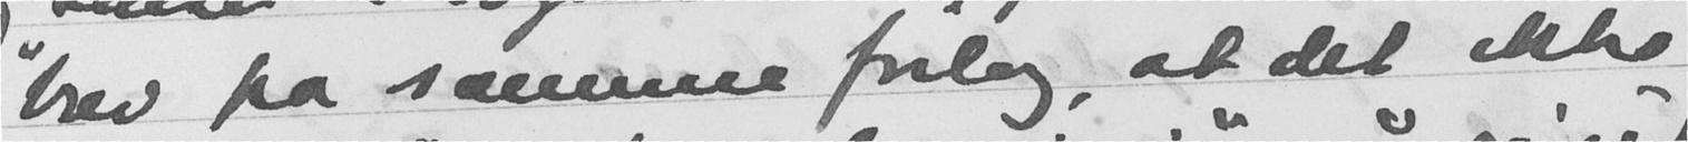brev fra samme forlag, at det ikke
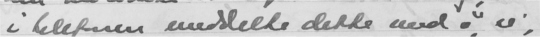i telefonen meddelte dette med å si,
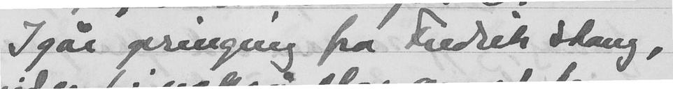Igår opringing fra Fredrik Stang,
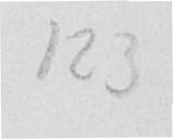125
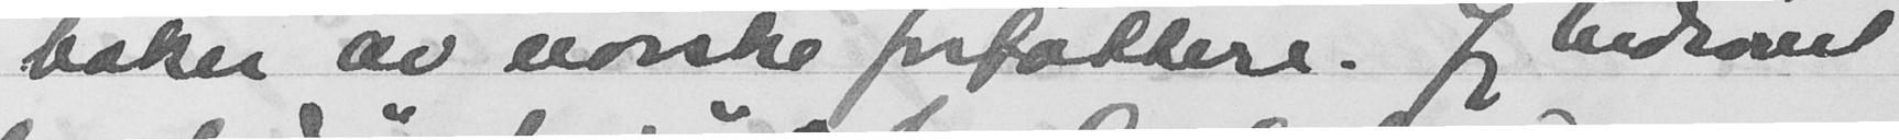bøker av norske forfattere. Jeg bedrøvet
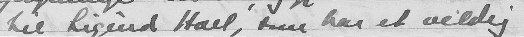til Sigurd Hoel, som har et veldig
